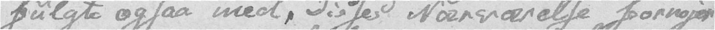fulgte ogsaa med, Disses Nærværelse fornøjer
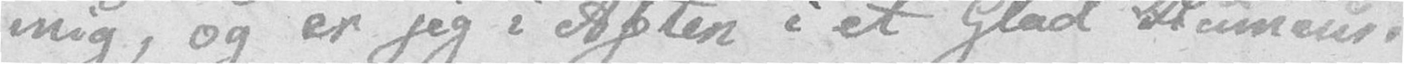mig, og er jeg i Aften i et Glad Humeur.
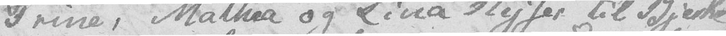Trine, Mathea og Lina Rejser til Bjerke
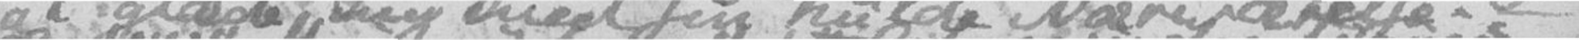at glæde, mig med sin hulde Nærværelse. –
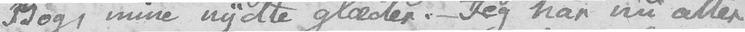Bog, mine nydte glæder. – Jeg har nu atter
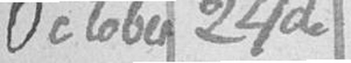October 24de
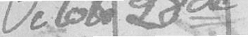Octobe 28de
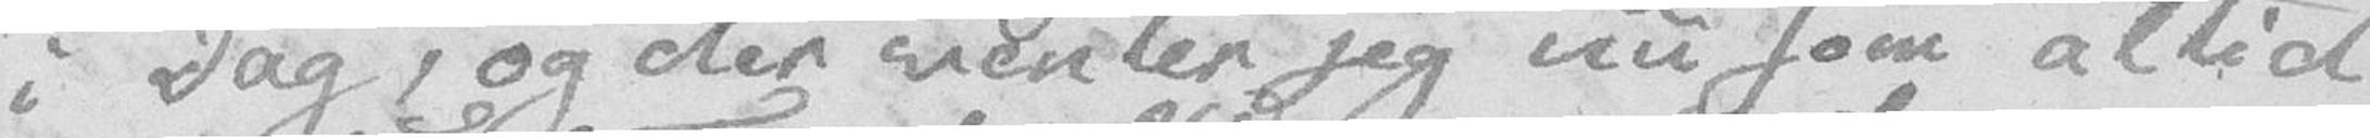i Dag, og der venter jeg nu som altid
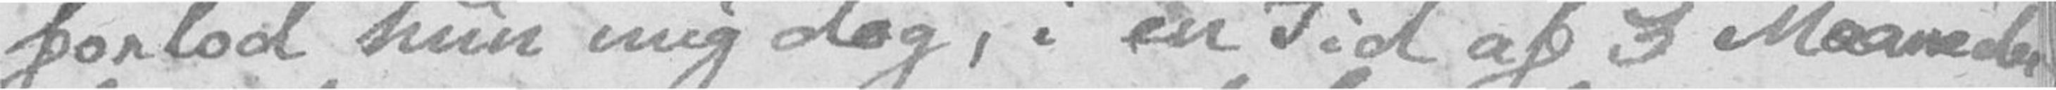forlod hun mig dog, i en Tid af 3 Maaneder
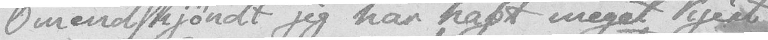Omendskjøndt jeg har haft meget kjert
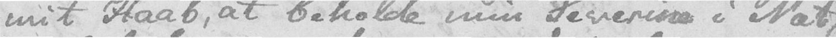mit Haab, at beholde min Severine i Nat,
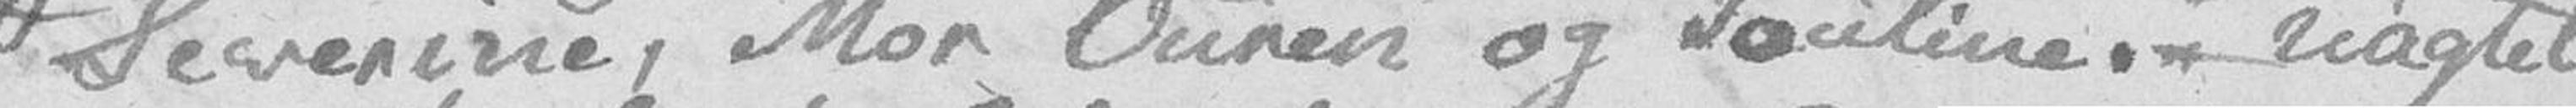Severine, Mor Ouren og Pouline. – Uagtet
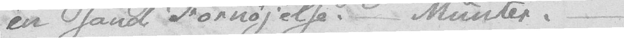en sand Fornøjelse. – Munter. –
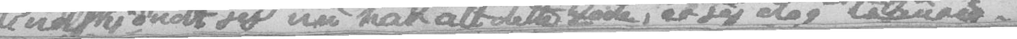Endskjøndt jeg nu har alt dette Gode, er jeg dog traurig.
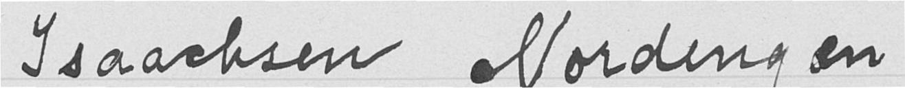Isaachsen Nordengen
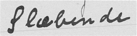Slæbende
